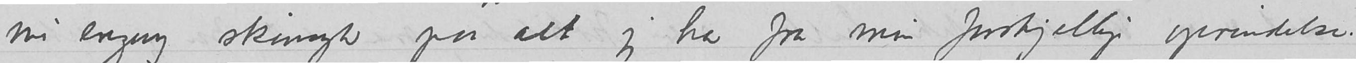nu engang skinsyk paa alt jeg har fra min(e) forskjellige oprindelser
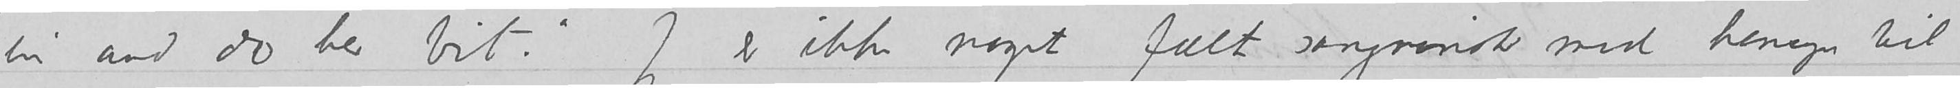in and do her bit.» Jeg er ikke noget fælt sangvinsk med hensyn til
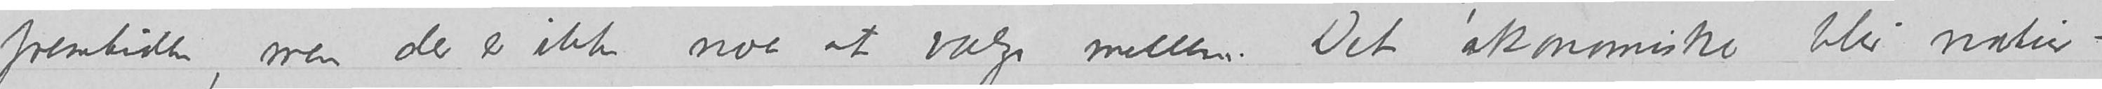fremtiden, men der er ikke noe at vælge mellem. Det økonomiske blir naturligvis
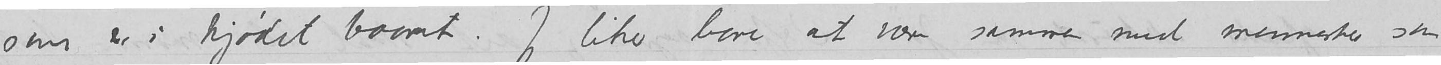som er i kjødet baaret. Jeg liker bare at være sammen med
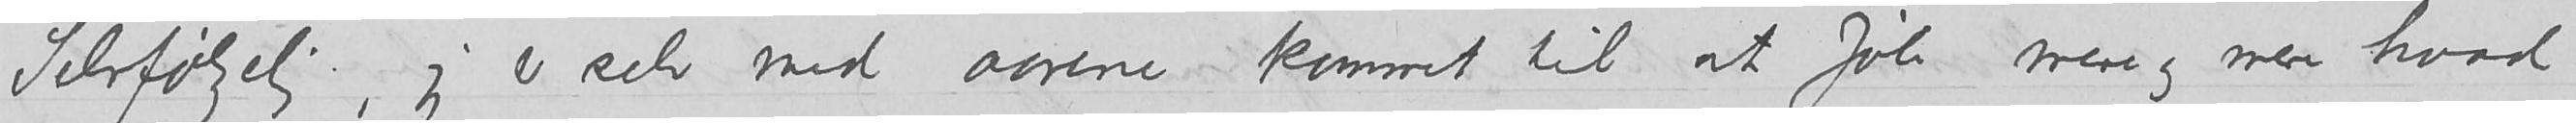Selvfølgelig, jeg er selv med aarene kommet til at føle mere og mere hvad
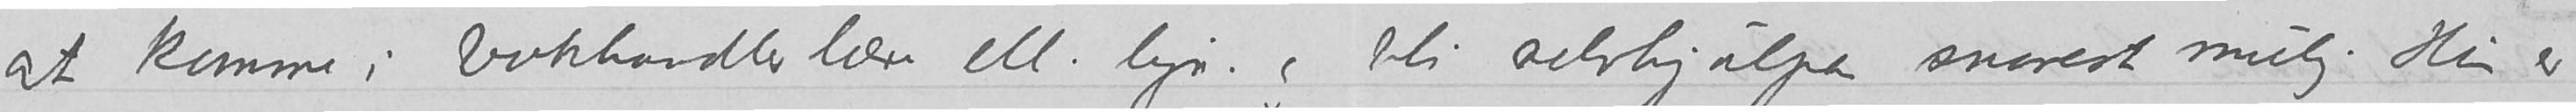at komme i bokhandlerlære ell. lign. og bli selvhjulpen snarest mulig. Hun er
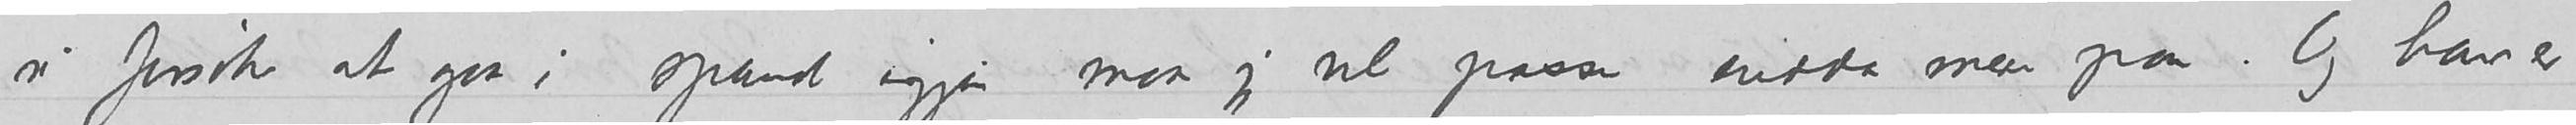vi forsøke at gaa i spand igjen maa jeg vel passe endda mere paa. Og han er
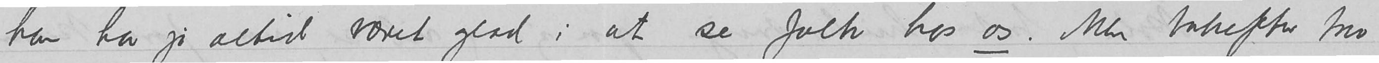, han har jo altid været glad i at se folk hos os.  Men bakefter tror
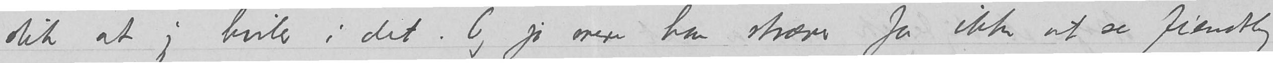slik at jeg hviler i det. Og jo mere han stræver for ikke at se fiendtlig
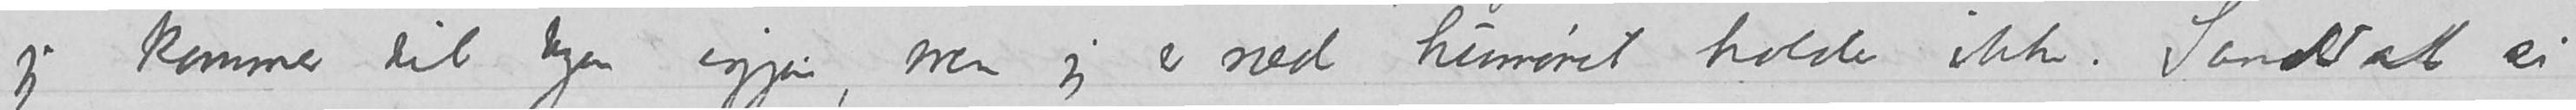jeg kommer til byen igjen, men jeg er ræd humøret holder ikke.  Sandt at si
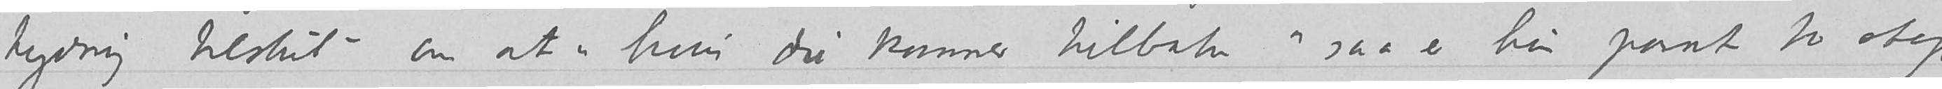tydning tilslut om at «hvis du kommer tilbake» saa er hun parat to step
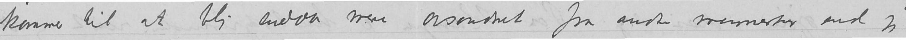kommer til at bli endda mere avsondret fra andre mennesker end jeg
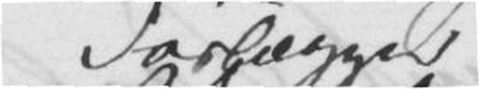Forlægger
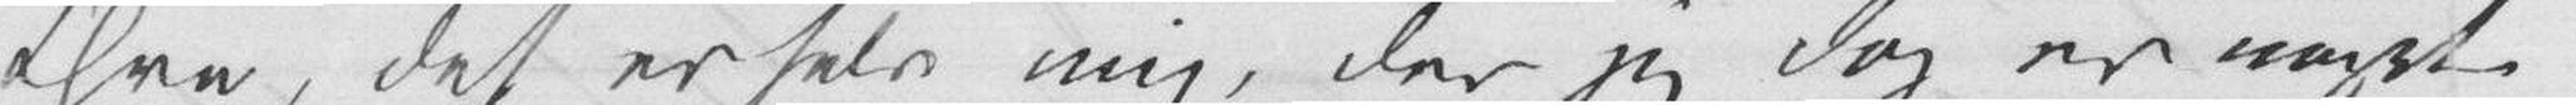Øre, det er selv mig, der jeg dog er noget
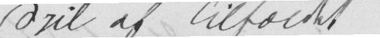Spil af Tilfældet
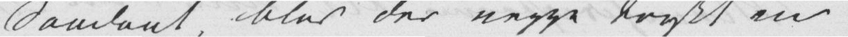Saadant, blev der neppe trykt en
